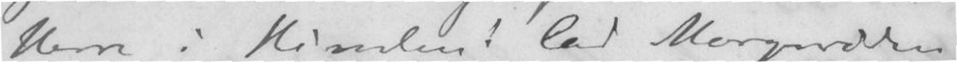Herre i Himlen! Lad Morgenrøden
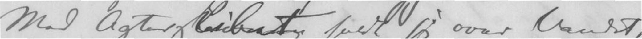Mod Agterskibet fuldt sø over Vandet,
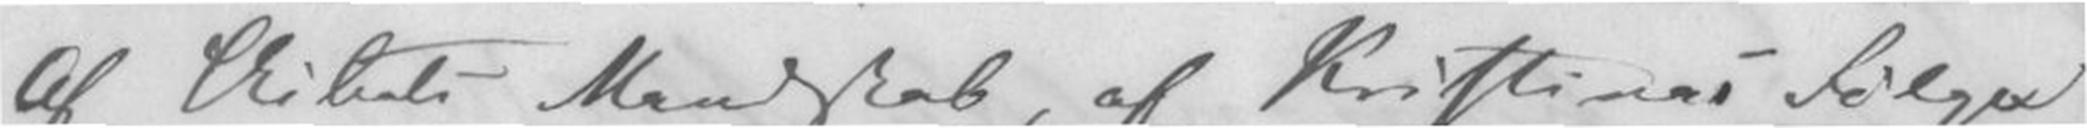Af Skibets Mandskab, af Kristinas Følge
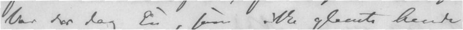Var der dog En, som ikke glemte hende,
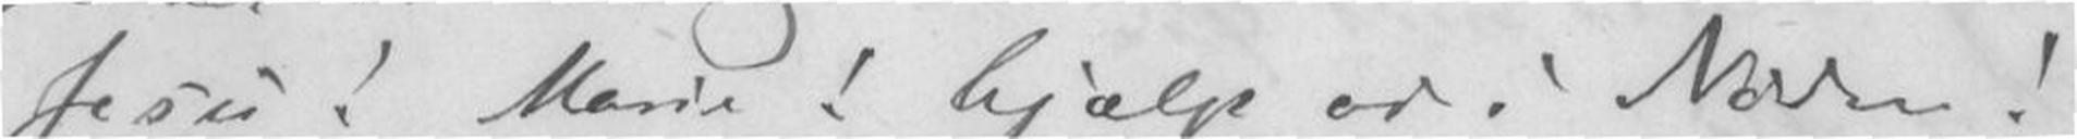Jesu! Maria! hjælpe os i Nøden!
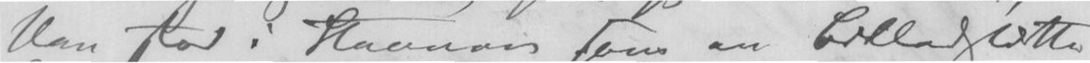Han stod i Stavnen som en Billedstøtte
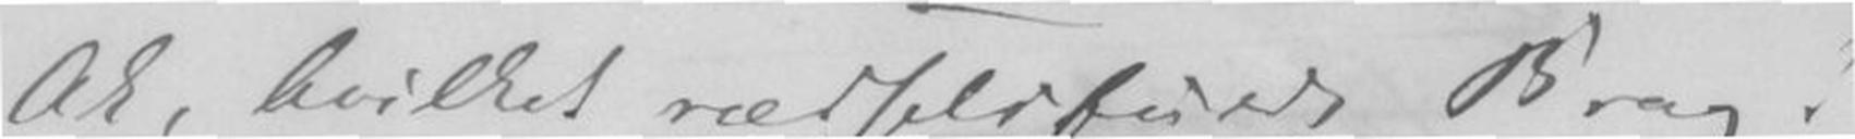Ak, hvilket rædselsfuldt Brag!
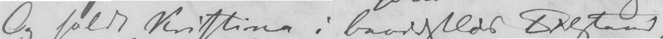Og fulde Kristina i baadestløs Tilstand
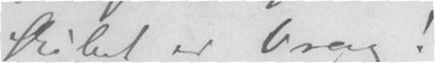Skibet er Vrag!
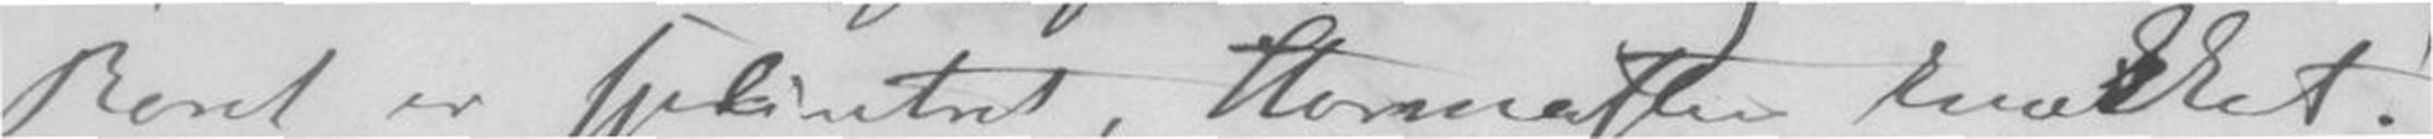Revet er splintret, stormasten knekket!
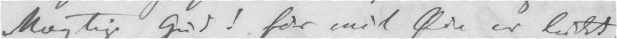Mægtige Gud! før mit Øie er lukket,
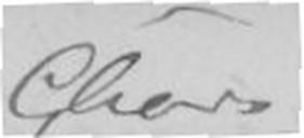Chor.
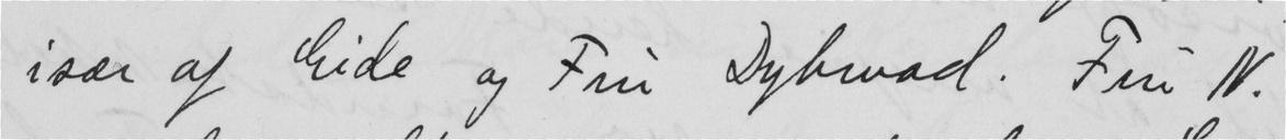især af Eide og Fru Dybwad. Fru N.
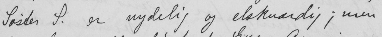Søster S. er nydelig og elskværdig; men
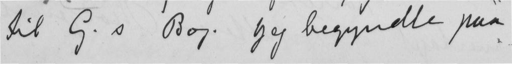til G.s. Bog. Jeg begyndte paa
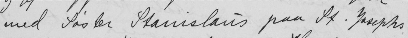med Søster Stamislaus paa St. Josephs
